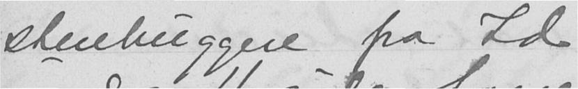stenhuggere fra Id.
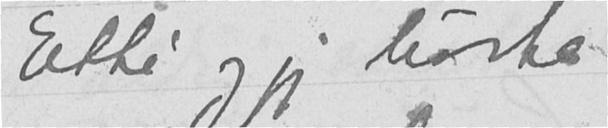Etti og jeg hørte
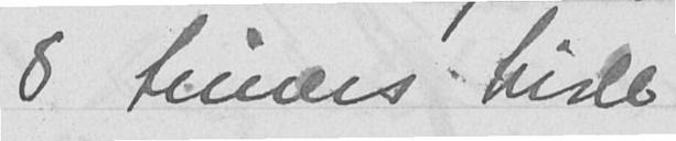8 timers hvile
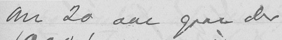Om 20 aar gaar der
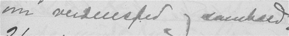om verdensfred og samhold.
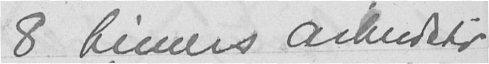8 timers arbeidstid
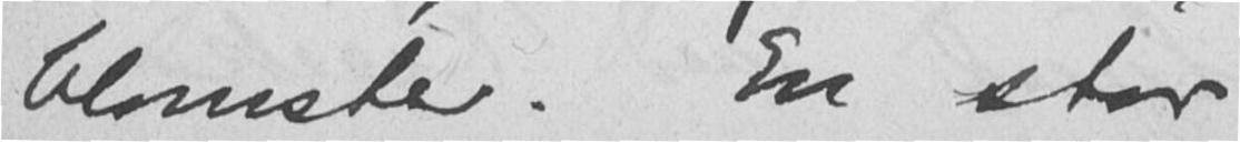blomster. En stor
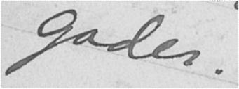gader.
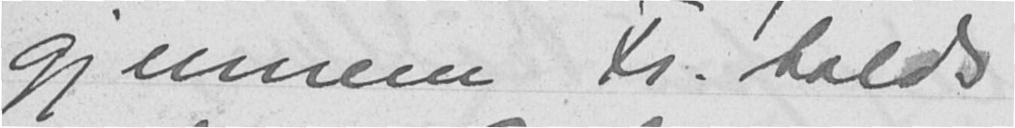gjennem Fr. halds
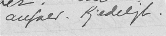anfald. Kjedeligt.
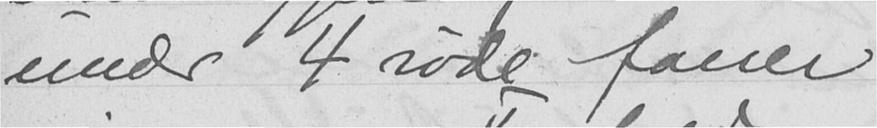under 4 røde faner
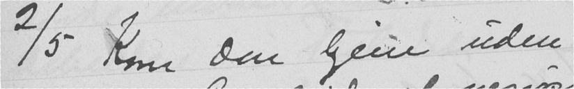2/5 Kom Don hjem uden
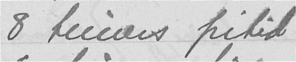8 timers fritid
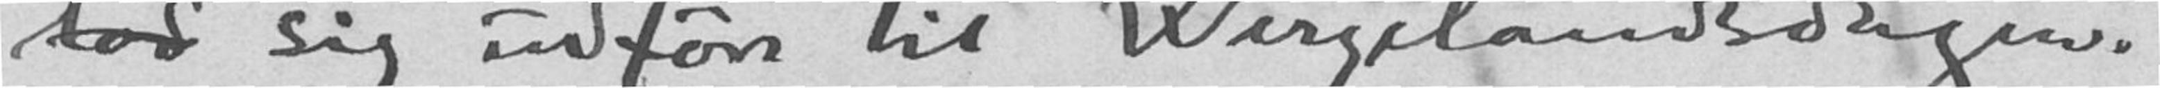lod sig udføre til Wergelandsdagen.
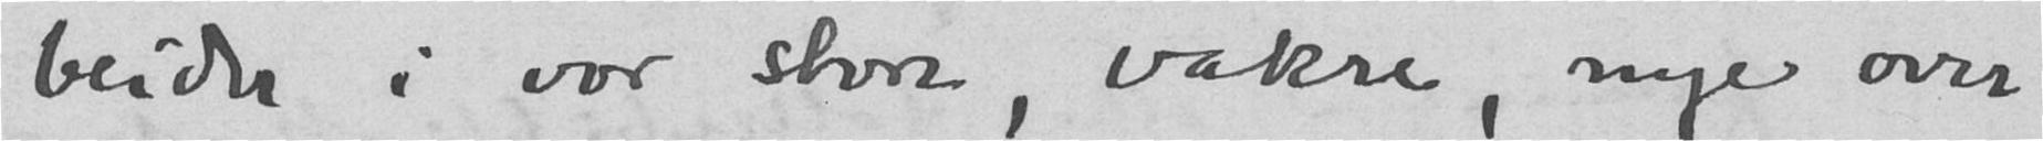beider i vor store, vakre, nye over-
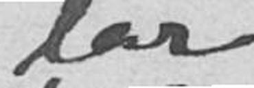lar
